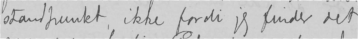standpunkt, ikke fordi jeg finder det
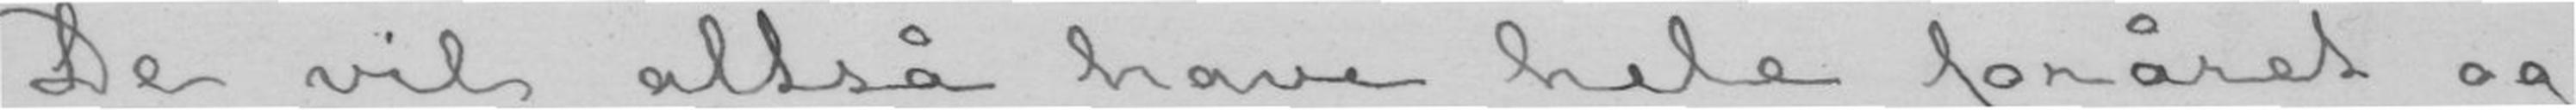De vil altså have hele foråret og
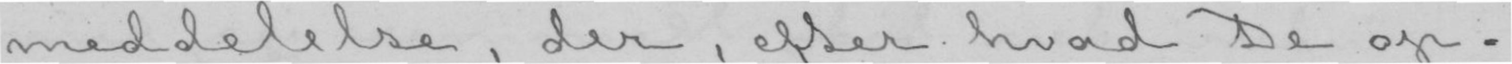meddelelse, der, efter hvad De op-
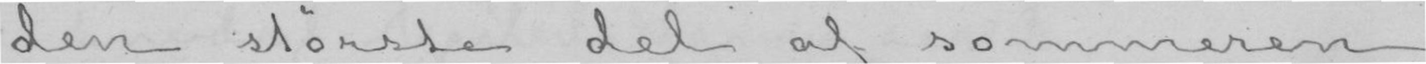den største del af sommeren
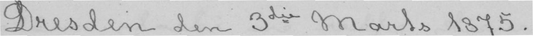Dresden den 3die Marts 1875.
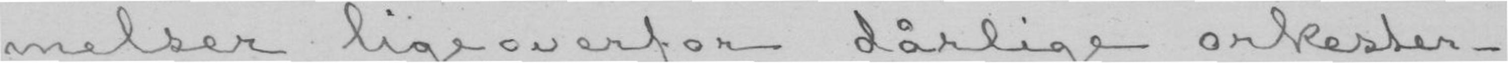melser ligeoverfor dårlige orkester-
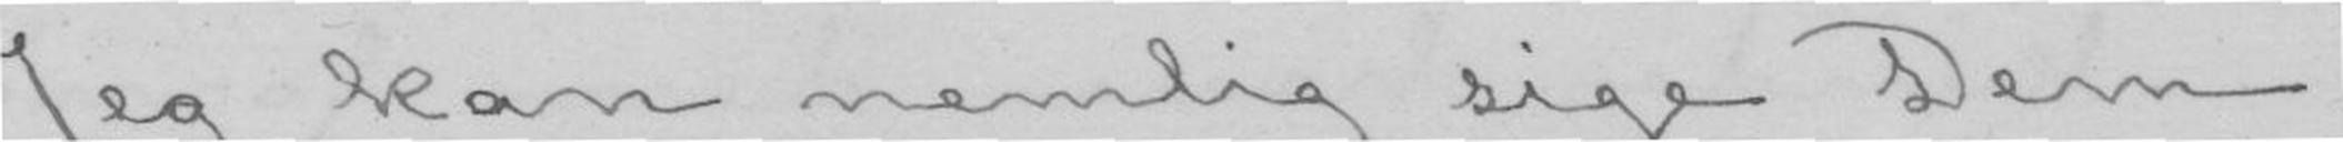Jeg kan nemlig sige Dem
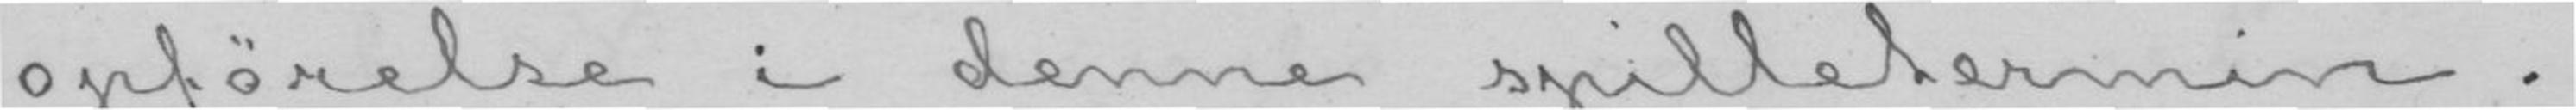opførelse i denne spilletermin.
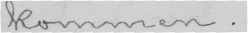kommen.
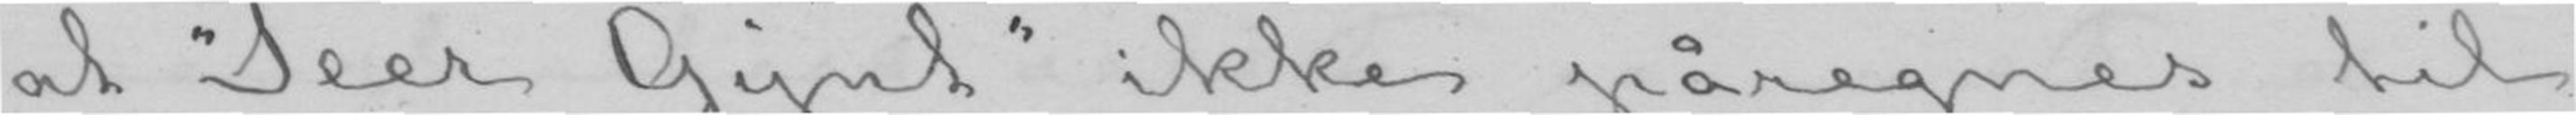at "Peer Gynt" ikke påregnes til
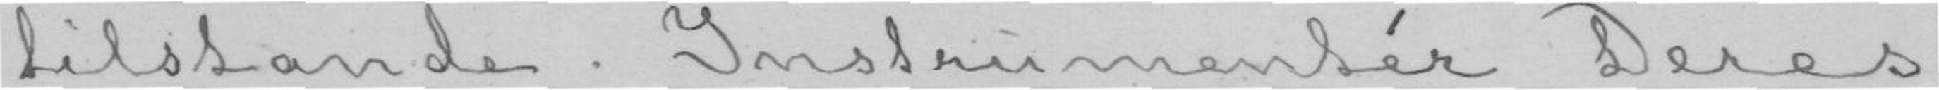tilstande. Instrumentér Deres
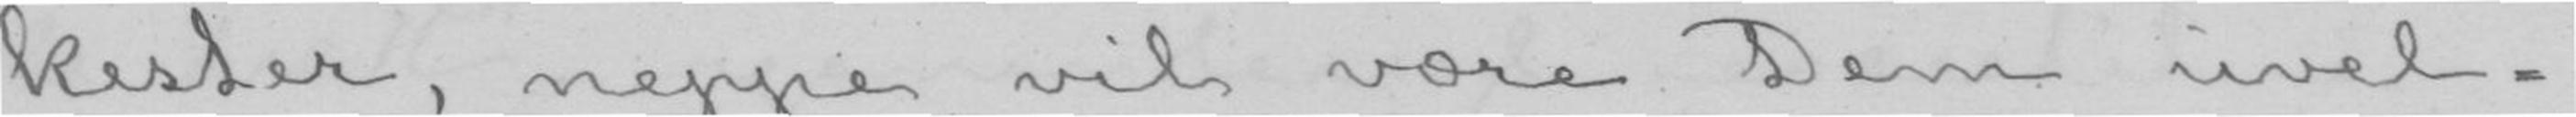kester, neppe vil være Dem uvel-
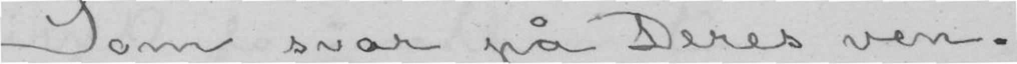Som svar på Deres ven-
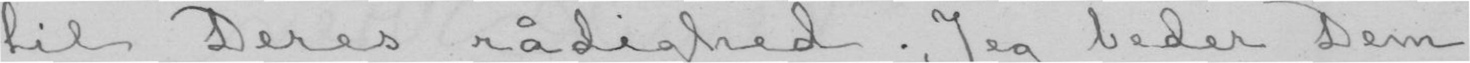til Deres rådighed. Jeg beder Dem
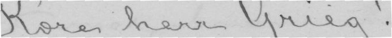Kære herr Grieg!
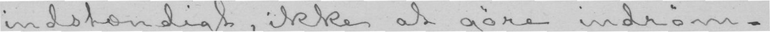indstændigt, ikke at gøre indrøm-
